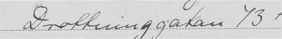Droftninggatan 73 A.
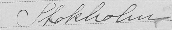Stokholm
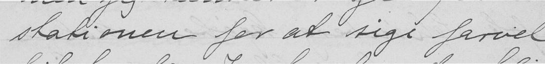stationen for at sige farvel
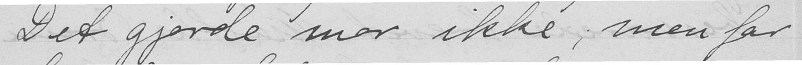Det gjorde mor ikke; men far
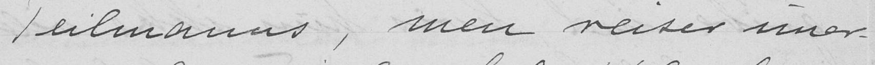Teilmanns, men reiser imor-
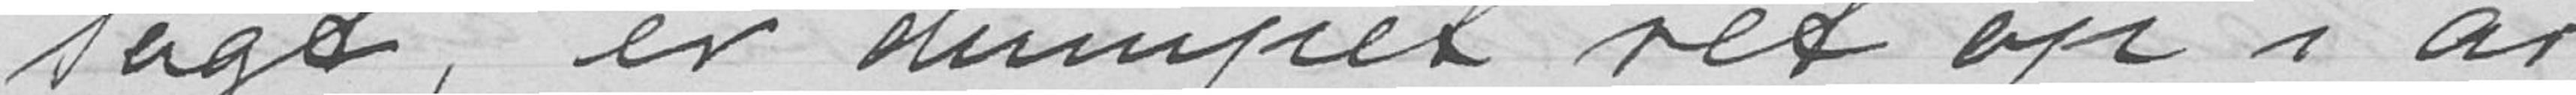sagt, er dumpet ret op i arbei-
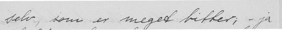selv, som er meget bitter; - ja
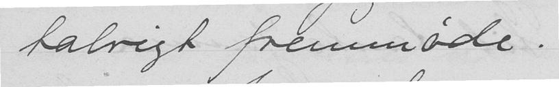talrigt fremmøde.
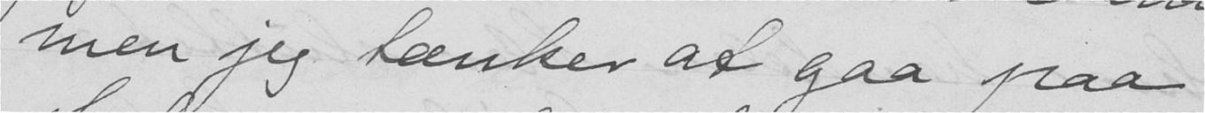men jeg tænker at gaa paa
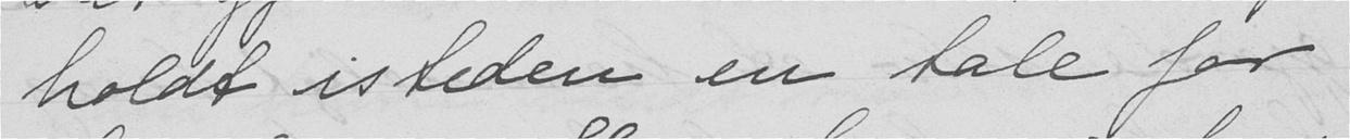holdt isteden en tale for
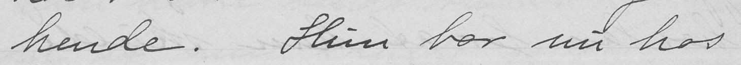hende. Hun bor nu hos
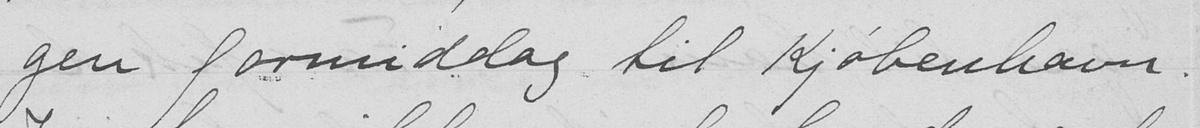gen formiddag til Kjøbenhavn.
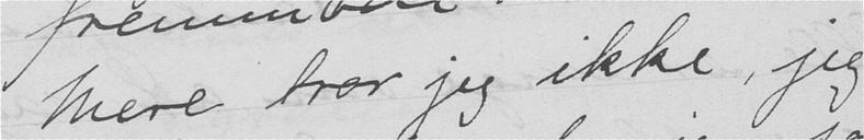Mere tror jeg ikke, jeg
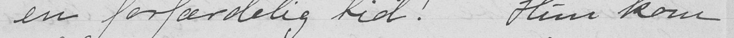en forfærdelig tid! Hun kom
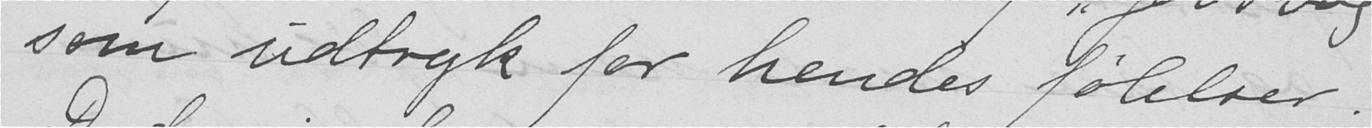som udtryk for hendes følelser.
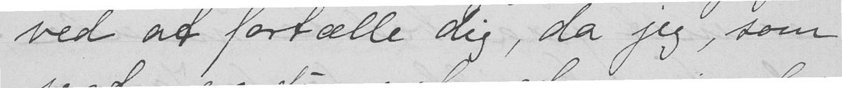ved at fortælle dig, da jeg, som
